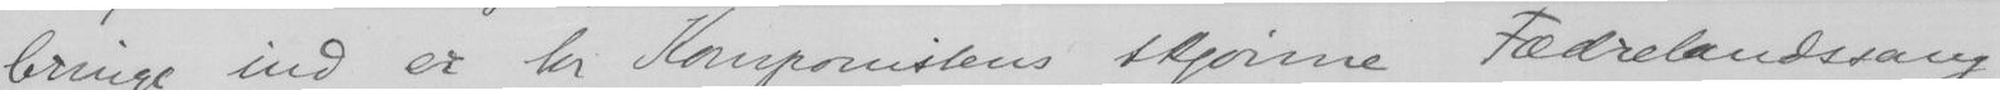bringe ind er Hr Komponistens skjønne Fædrelandssang
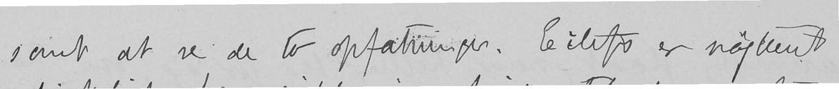somt at se de to opfatninger. Eilifs er nøgternt
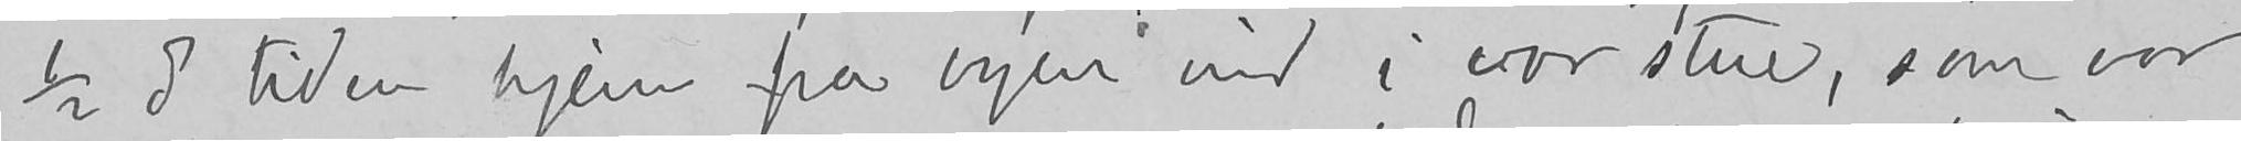1/2 5 tiden hjem fra byen ind i vor stue, som var
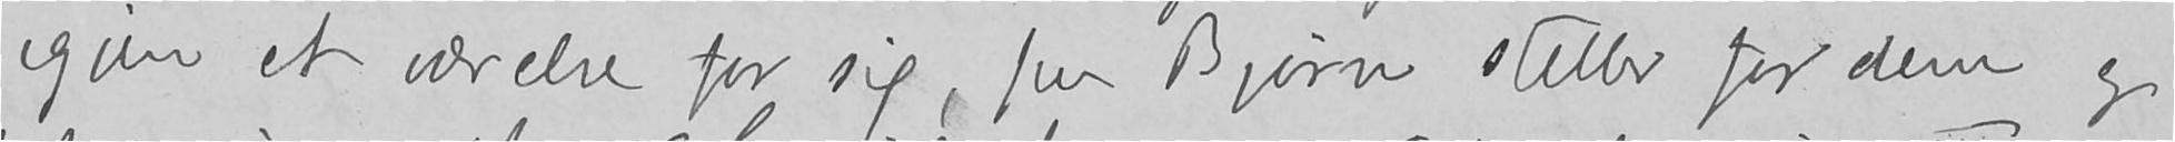igjen et værelse for sig, fru Bjørn steller for dem og
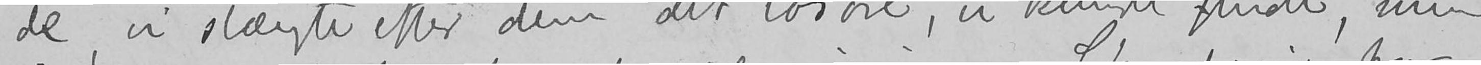de, vi slængte efter dem, det løsøre, vi kunde finde, men
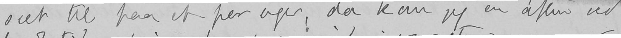seet til paa et par uger, da kom jeg en aften ved
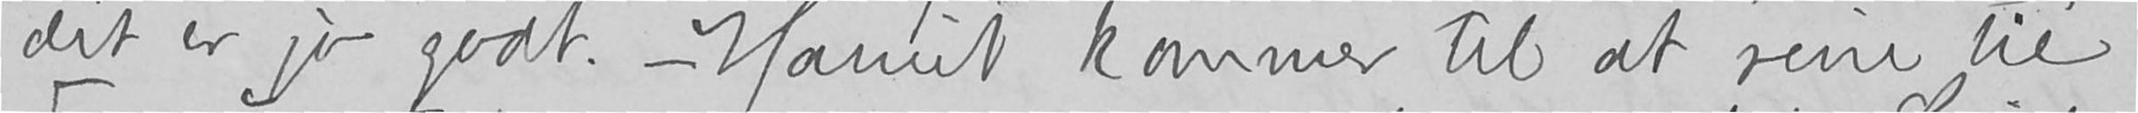det er jo godt. - Harriet kommer til at reise til
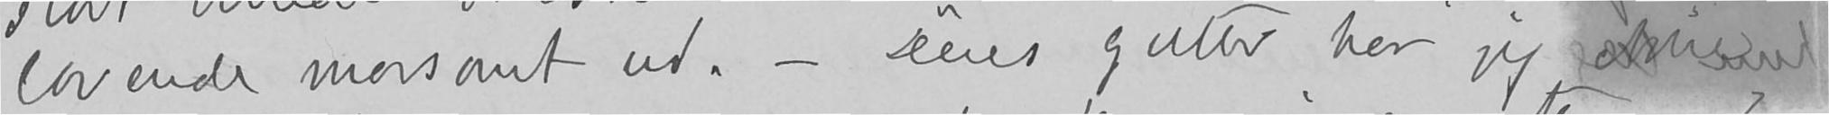lovende morsomt ud. - Deres gutter har jeg ikke
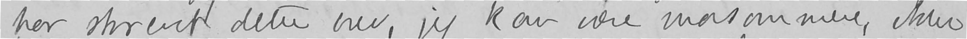har skrevet dette brev, jeg kan være morsommere, ikke
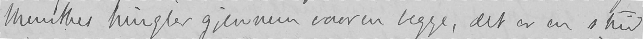Munthes tingler gjennem vaaren begge, det er en strid
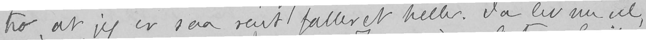tro, at jeg er saa rent falleret heller. Ja lev nu vel,
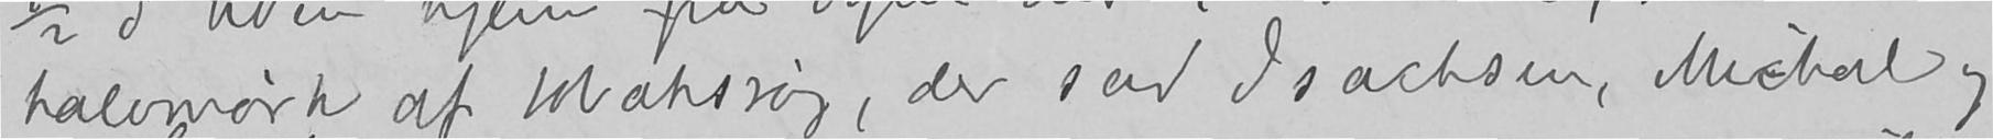halvmørk af tobaksrøg, der sad Isachsen, Michael og
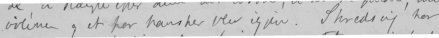violinen og et par hansker blev igjen. Skredsvig har
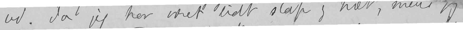ud. Ja jeg har været lidt slap og træt, mens jeg
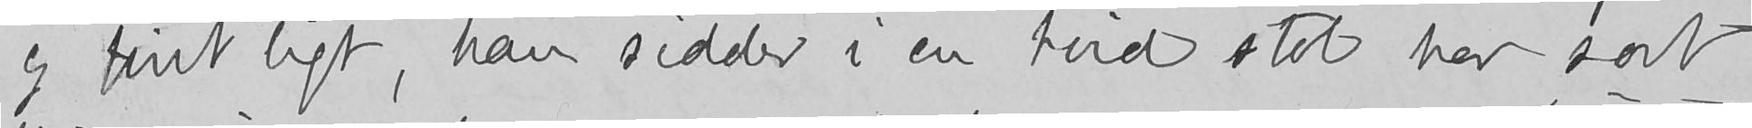og fint ligt, han sidder i en hvid stol har sort
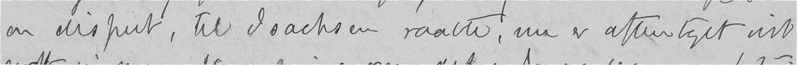en disput, til Isachsen raabte, nu er aftentoget vist
